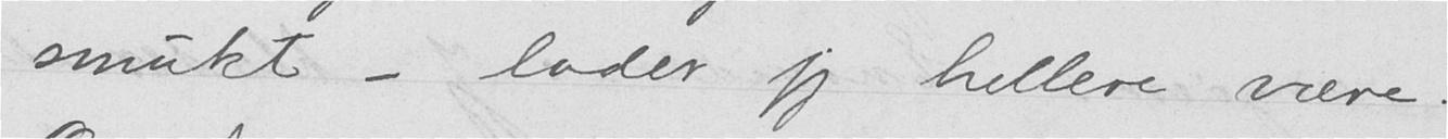smukt - lader jeg hellere være.
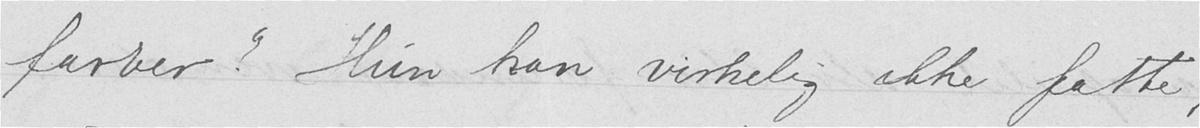farver"! Hun kan virkelig ikke fatte,
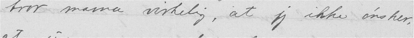tror mama virkelig, at jeg ikke ønsker,
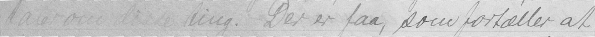taler om disse ting. Der er faa, som fortæller at
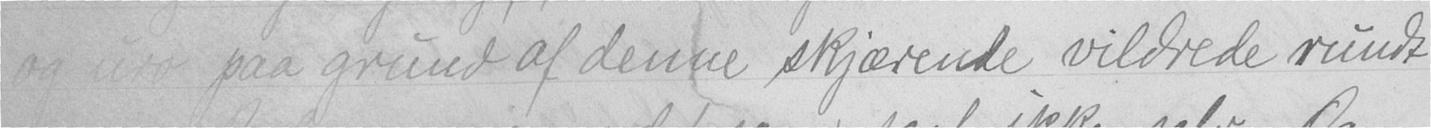og uro paa grund af denne skjærende vildrede rundt
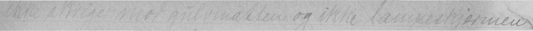ikke skriger mod gulvmatten og ikke lampeskjermen
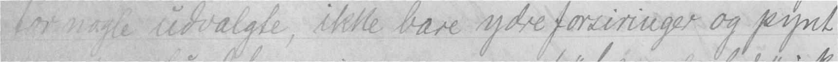for nogle udvalgte, ikke bare ydre forsiringer og pynt
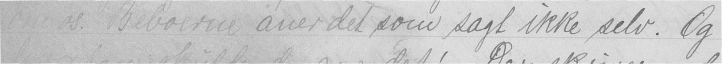om os. Beboerne aner det som sagt ikke selv. Og
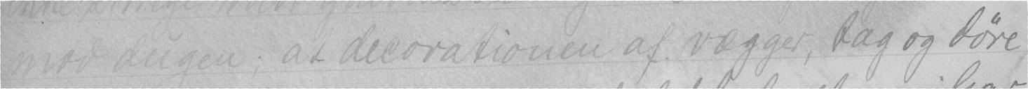mod dugen; at decorationen af vægger, tag og døre
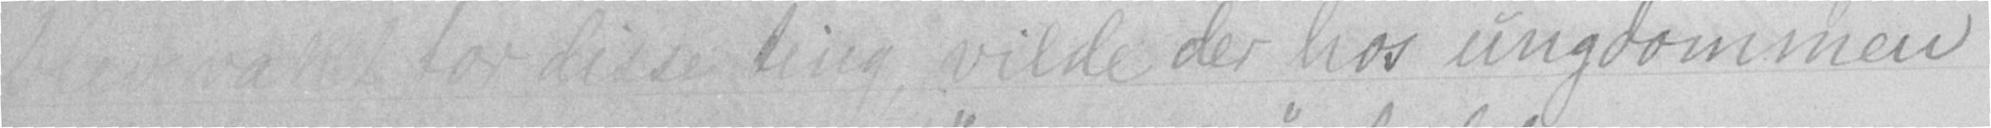blev vakt for disse ting, vilde der hos ungdommen
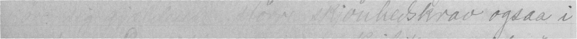skjønhedskrav ogsaa i
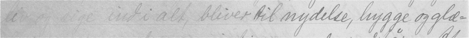liv og sige ind i alt, bliver til nydelse, hygge og glæ-
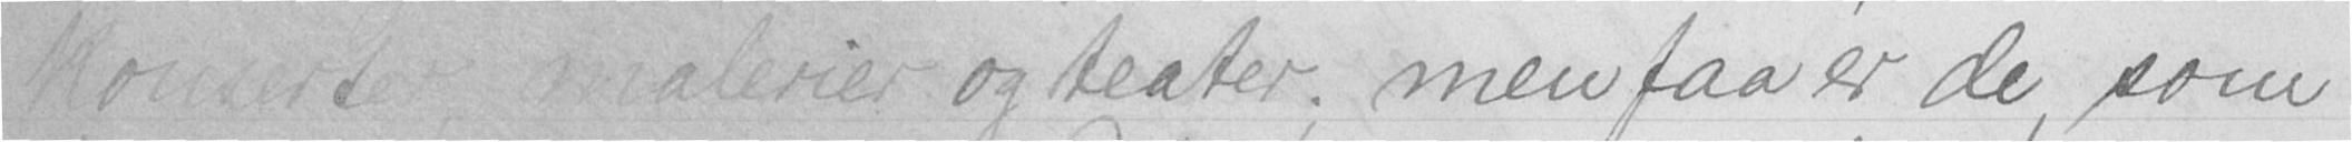konserter, malerier og teater; men faa er de, som
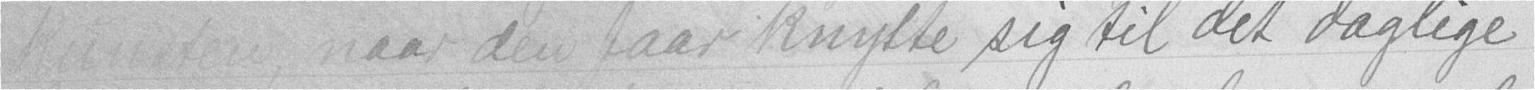kunsten, naar den faar knytte sig til det daglige
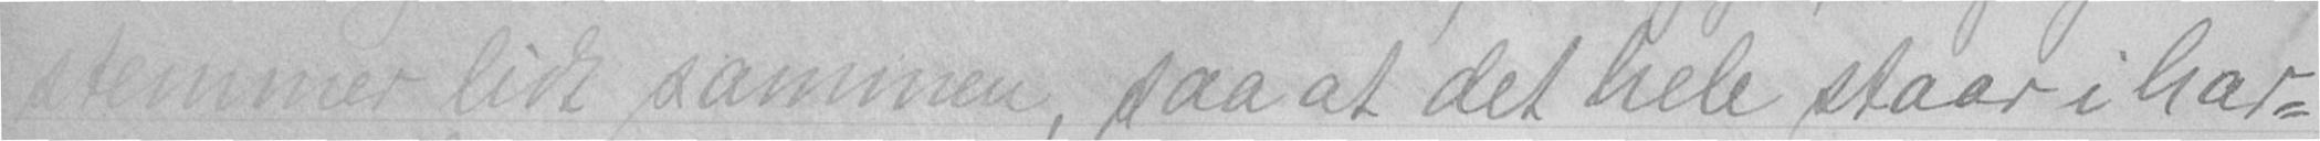stemmer lidt sammen, saa at det hele staar i har-
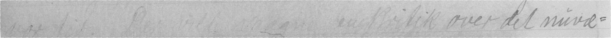vor tid. Der vilde   den kritik over det nuvæ-
